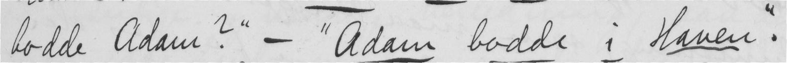bodde Adam?" — "Adam bodde i Haven".
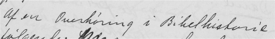Af en Overhøring i Bibelhistorie
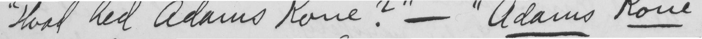"Hval hed Adams Kone?" - "Adams Kone
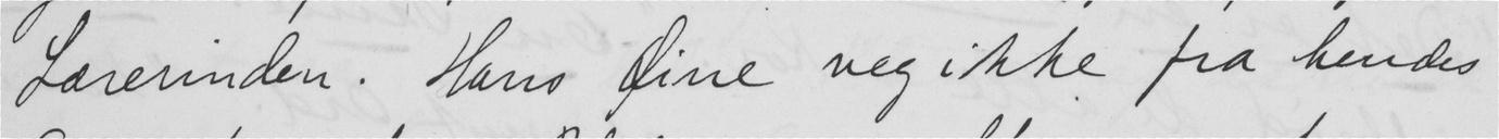Lærerinden. Hans Øine veg ikke fra hendes
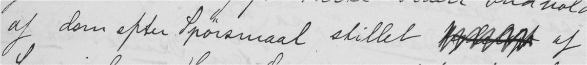af dem efter Spørsmaal stillet  af
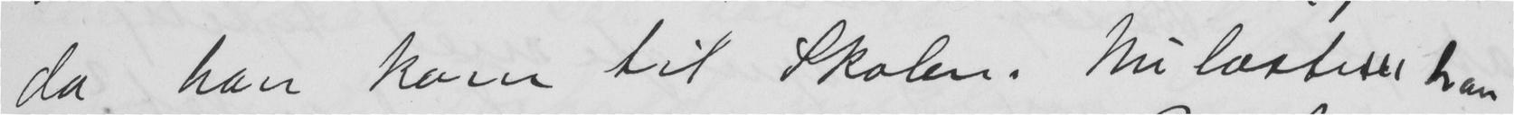da han kom til Skolen. Nu læste han
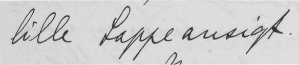lille Lappeansigt!
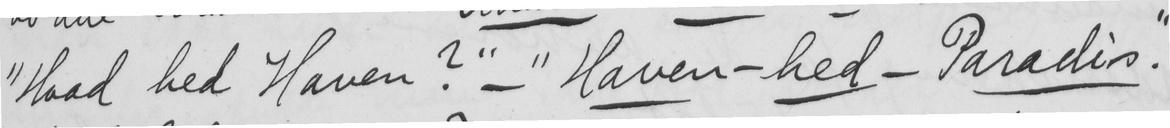"Hvad hed Haven?" - "Haven - hed - Paradis".
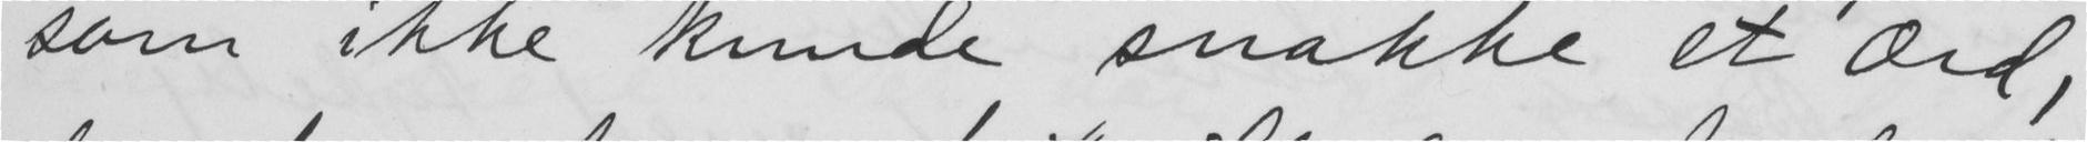som ikke kunde snakke et Ord,
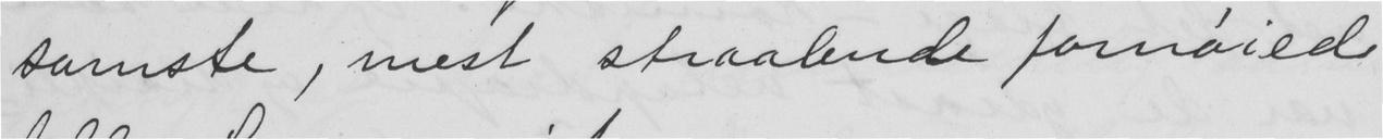samste, mest straalende fornøiede
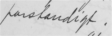forstandigt.
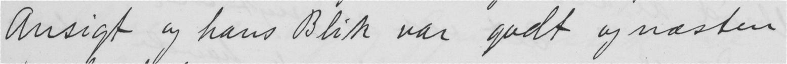Ansigt og hans Blik var godt og næsten
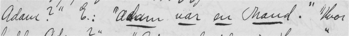Adam?" E.: "Adnm var en Mand." "Hvor
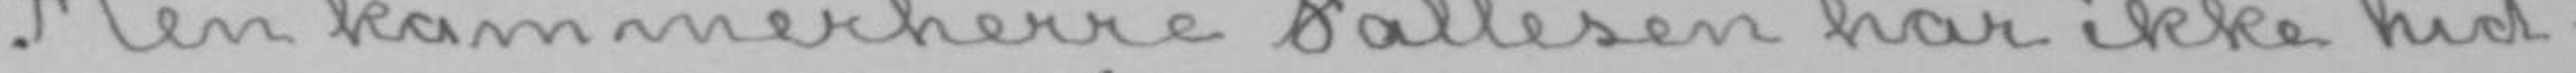Men kammerherre Fallesen har ikke hid-
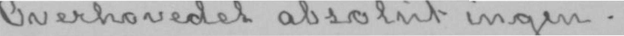Overhovedet absolut ingen.-
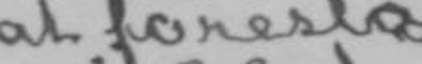at foreslå
